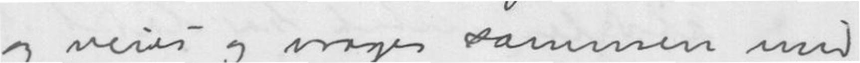og veies og vrages sammen med
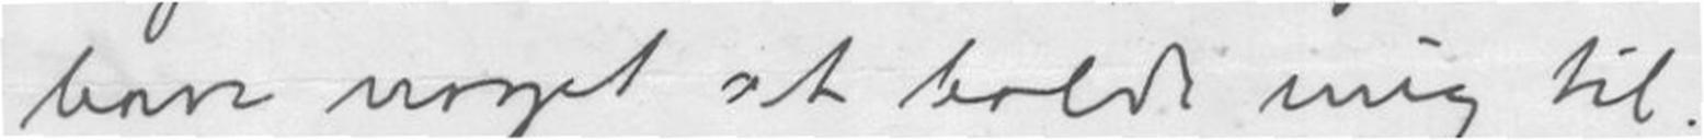have noget at holde mig til.
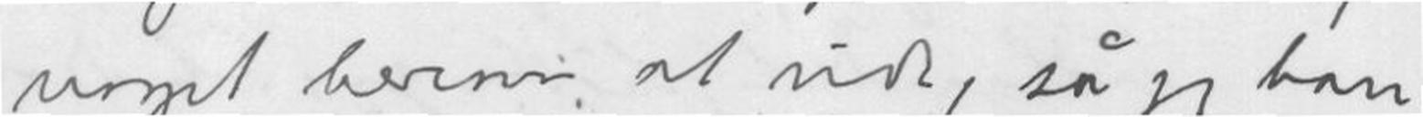noget herom at vide, så jeg kan
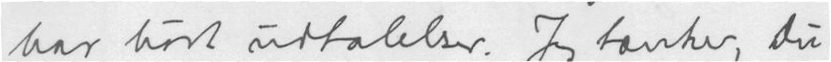har hørt udtalelser. Jeg tænker, du
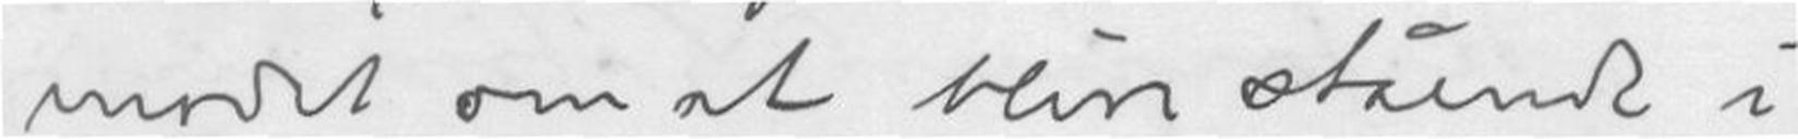modet om at blive stående i
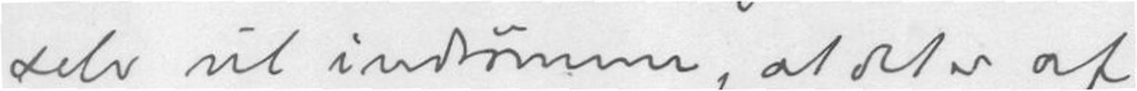selv vil indrømme, at det er af
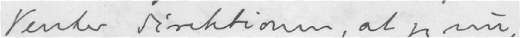Venter direktionen, at jeg nu,
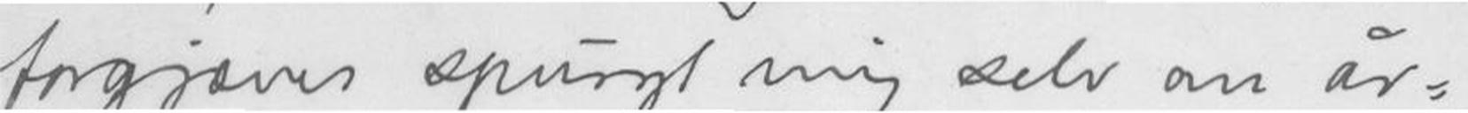forgjæves spurgt mig selv om år-
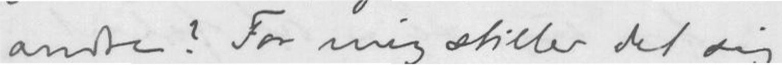andre? For mig stiller det sig
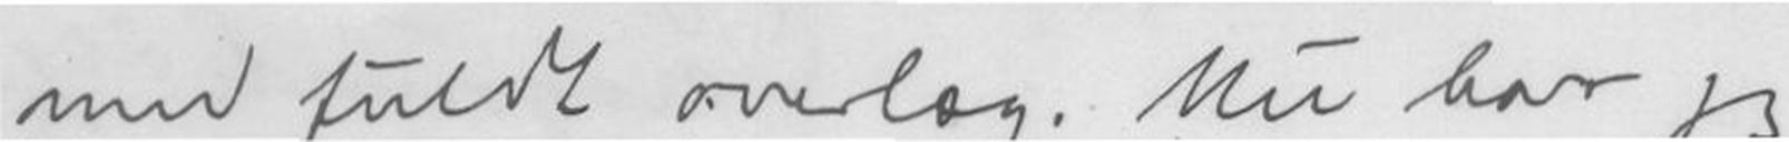med fuldt overlag. Nu har jeg
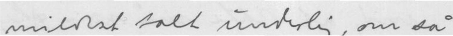mildest talt underlig, om så
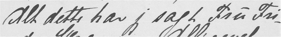Alt dette har jeg sagt Fru Fri-
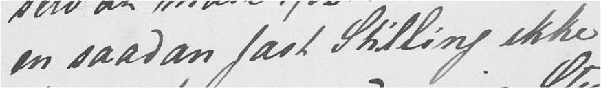en saadan fast Stilling ikke
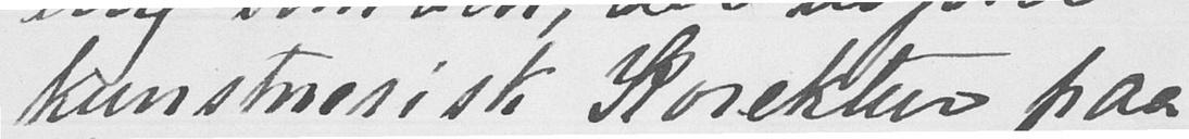kunstnerisk Korektur paa
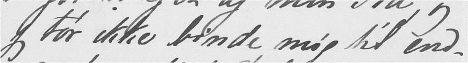jeg tør ikke binde mig til end-
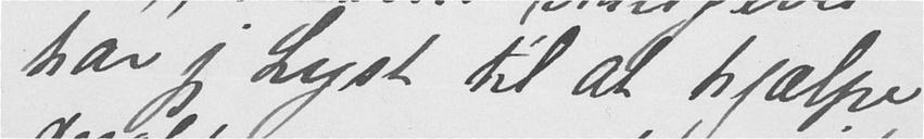har jeg Lyst til at hjælpe
